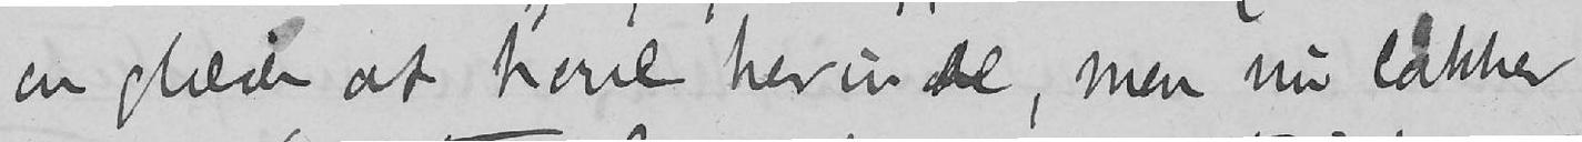en glæde at have herinde, men nu lakker
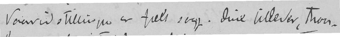Vaarudstillingen er fælt svag. Dine billeder, Thou-
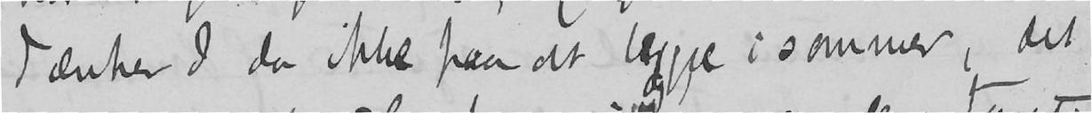Tænker I da ikke paa at bygge isommer, det
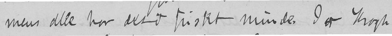mens alle har det i friskt minde. Især Krogh
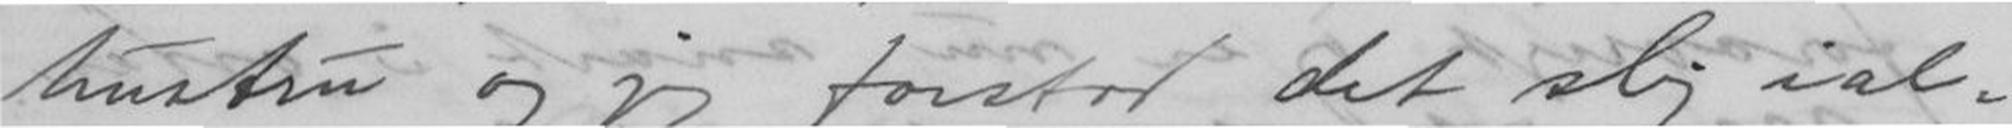hustru og jeg forstod det slig ial-
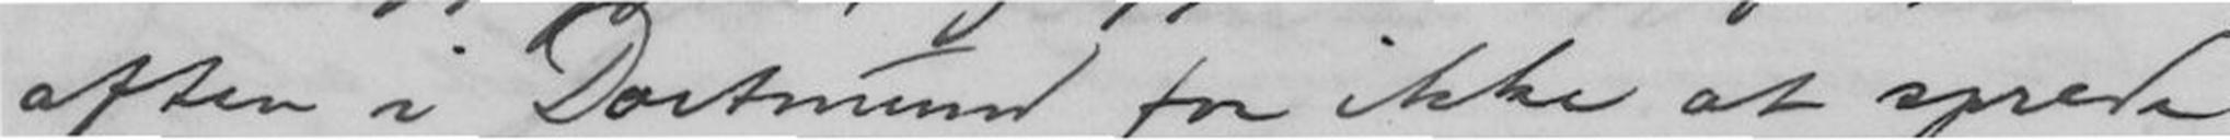aften i Dortmund for ikke at sprede
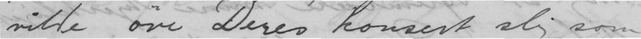vilde øve Deres konsert slig som
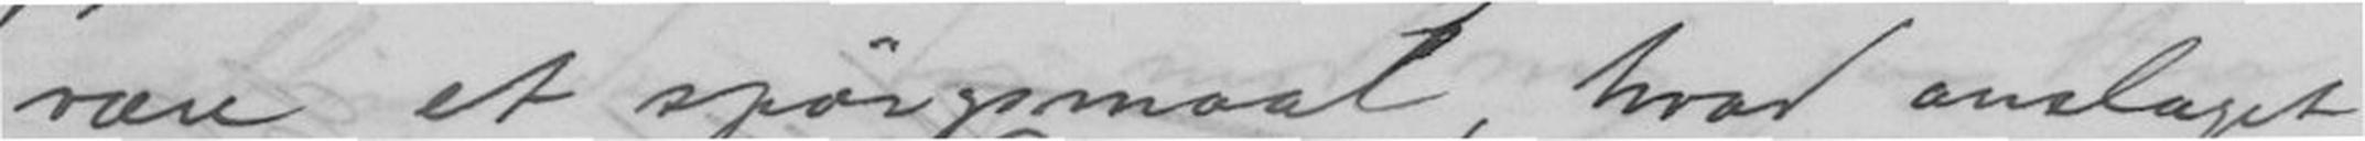være et spørgsmaal, hvad anslaget
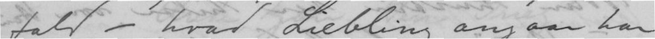fald, - hvad Liebling angaar har
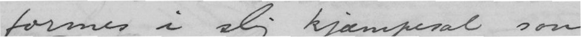formes i slig kjæmpesal som
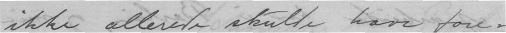ikke allerede skulde have fore-
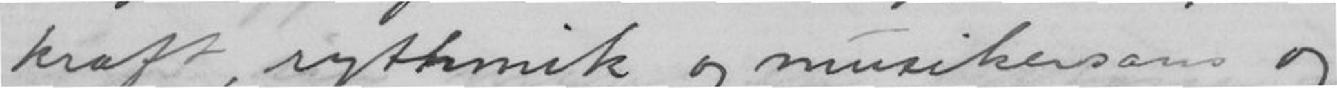kraft, rythmik og musikersans og
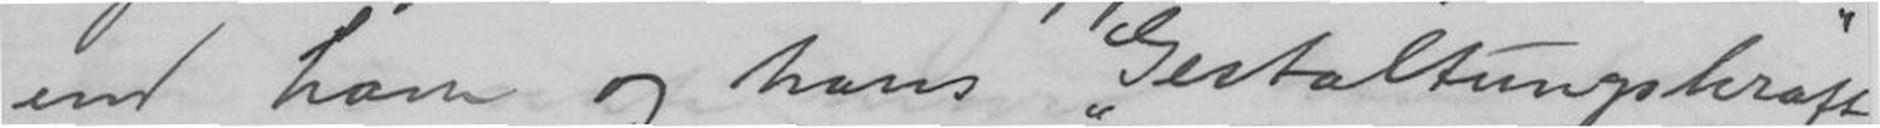end ham og hans "Gestaltungskraft"
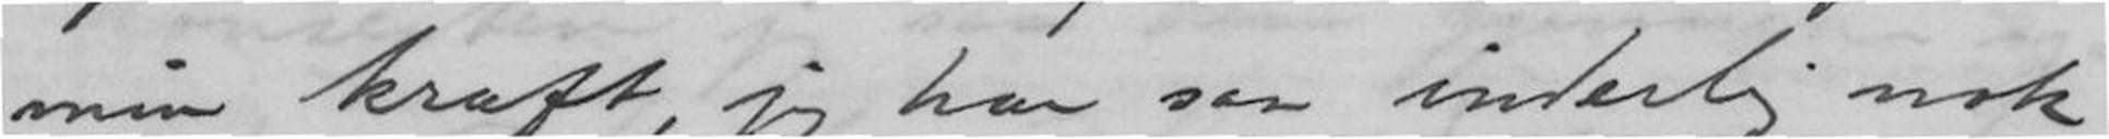min kraft, jeg har saa inderlig nok
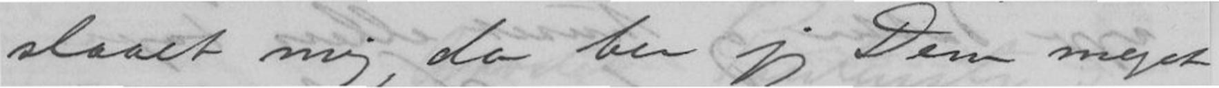slaaet mig, da ber jeg Dem meget
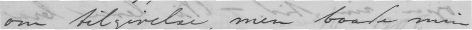om tilgivelse, men baade min
